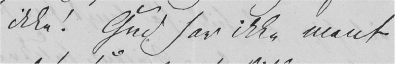ikke! Gud har ikke ment
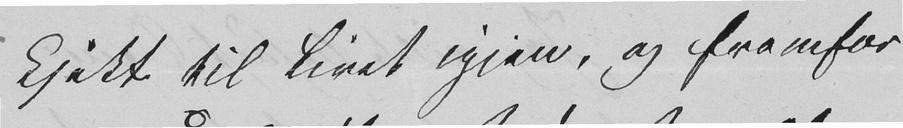kjekt til Livet igjen, og fremfor
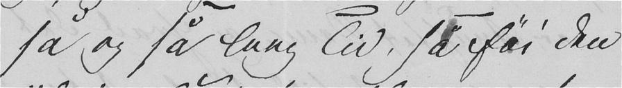så og så lang Tid, så føi den
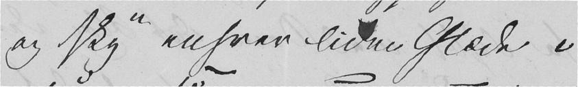og sky enhver liden Glæde i
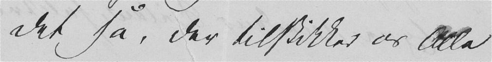det så, der tilskikker os Alle
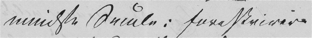mindste Smule: foreskriver
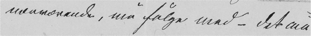nærværende, må følge med – det må
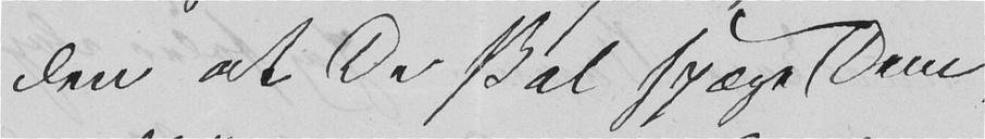den at De skal spæge Dem,
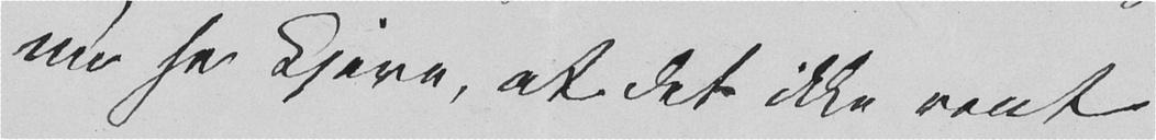nu se kjere, at det ikke rent
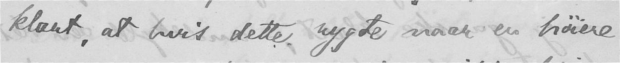klart, at hvis dette rygte naar en høiere
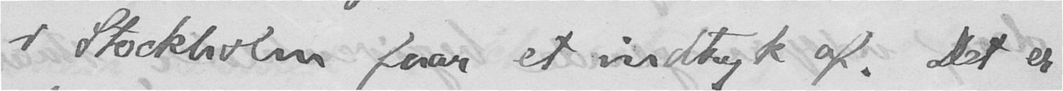i Stockholm faar et indtryk af. Det er
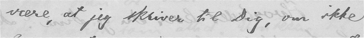være, at jeg skriver til Dig, om ikke
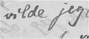vilde jeg
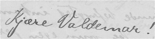Kjære Valdemar!
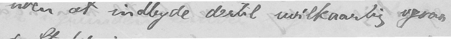uden at indbyde dertil uvilkaarlig ogsaa
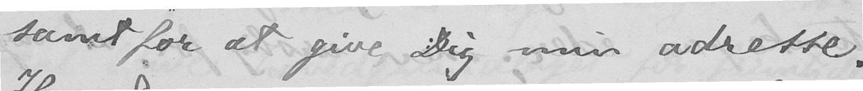samt for at give Dig min adresse.
